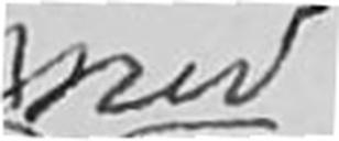med
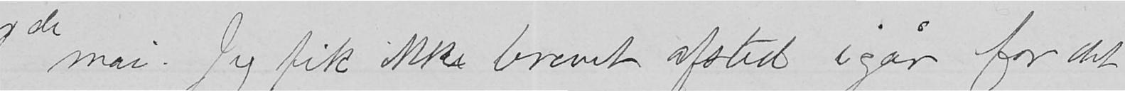mai. Jeg fik ikke brevet afsted igår for det
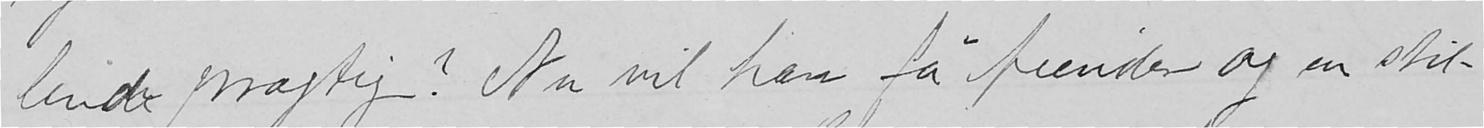lende prægtig? Nu vil han få fiender og en stil-
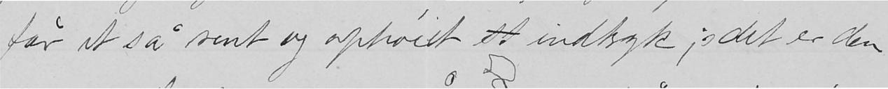får et så rent og ophøiet et indtryk; det er den
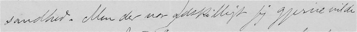sandhed. Men der var adskilligt jeg gjerne vilde
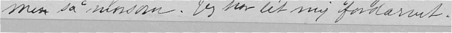men så morsom. Jeg har lét mig fordærvt.
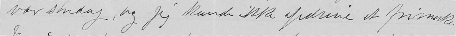var søndag, og jeg kunde ikke opdrive et frimærke.
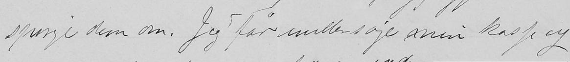spørge dem om. Jeg får undersøge min kasse og
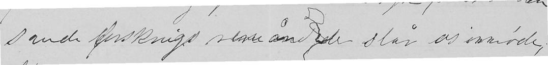sande forsknings rene ånd de slår os imøde,
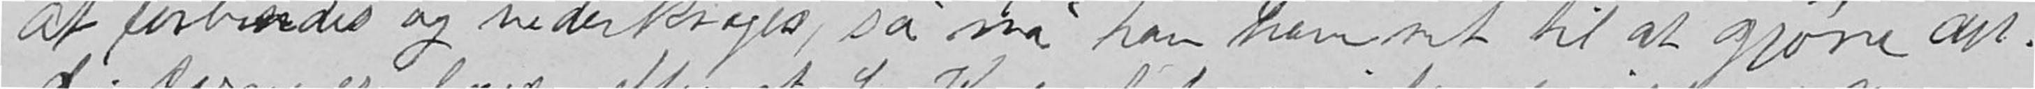at forbindes og vederkvæges, sa må han have ret til at gjøre det.
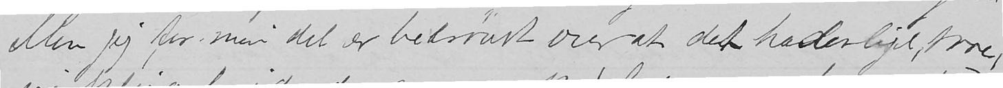Men jeg for min del er bedrøvet over at det hederlige, store,
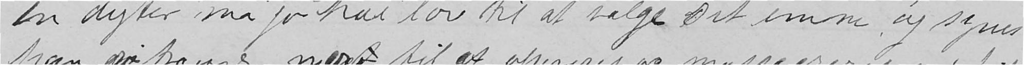en digter må jo hae' lov til at vælge sit emne, og synes
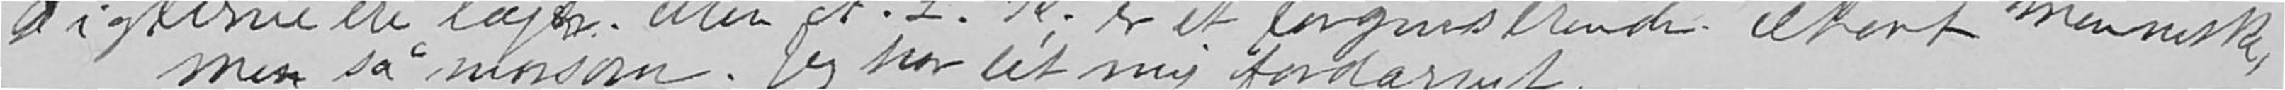Digterne ere læger. Men A.L.K. er et forgnistrende iltert menneske,
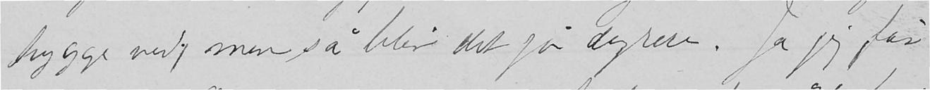hygge ved, men så blir det jo dyrere. Ja jeg får
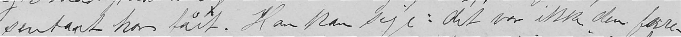sentant har fået. Han kan sige: det var ikke den fore-
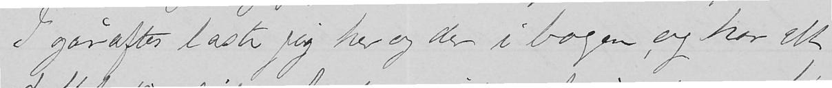I gåraftes læste jeg her og der i bogen, og har ett
